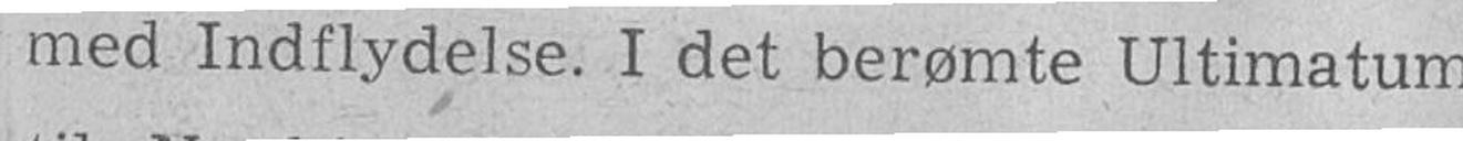med Indflydelse. I det berømte Ultimatum
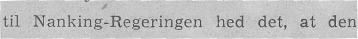til Nanking-Regeringen hed det, at den
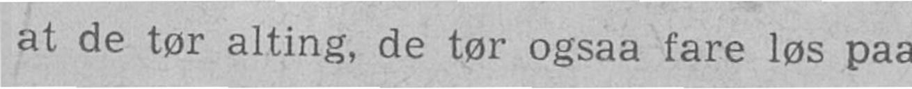at de tør alting, de tør ogsaa fare løs paa
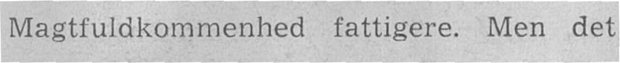Magtfuldkommenhed fattigere. Men det
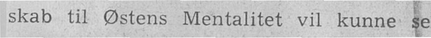skab til Østens Mentalitet vil kunne se
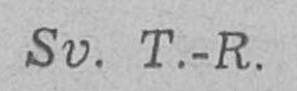Sv. T.-R.
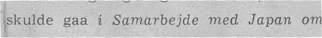skulde gaa i Samarbejde med Japan om
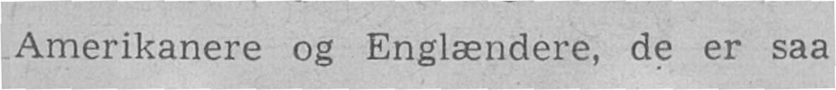Amerikanere og Englændere, de er saa
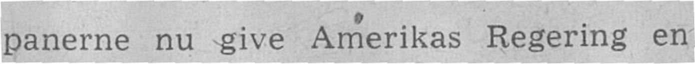panerne nu give Amerikas Regering en
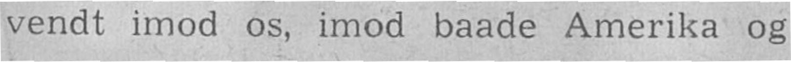vendt imod os, imod baade Amerika og
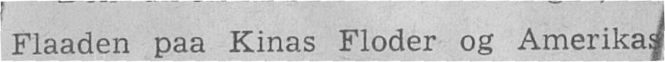Flaaden paa Kinas Floder og Amerikas
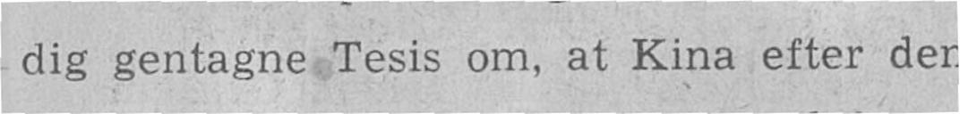dig gentagne Tesis om, at Kina efter den
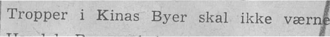Tropper i Kinas Byer skal ikke værne
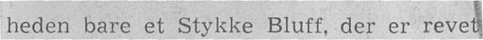heden bare et Stykke Bluff, der er revet
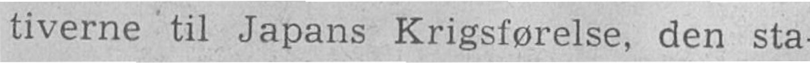tiverne til Japans Krigsførelse, den sta-
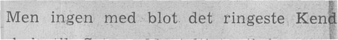Men ingen med blot det ringeste Kend-
